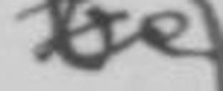be
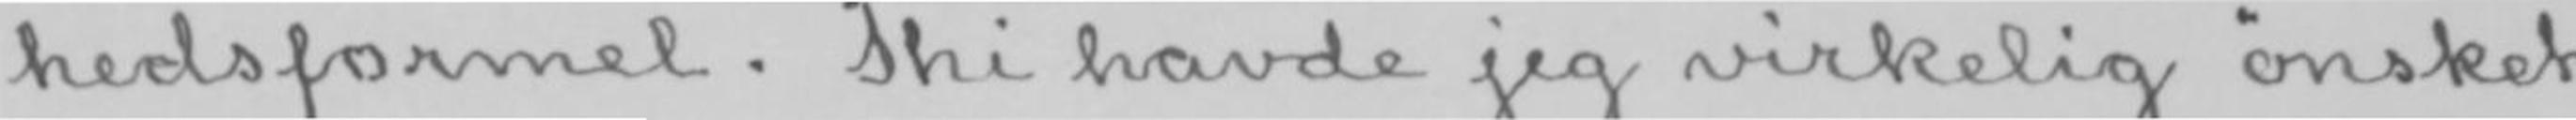hedsformel. Thi havde jeg virkelig ønsket
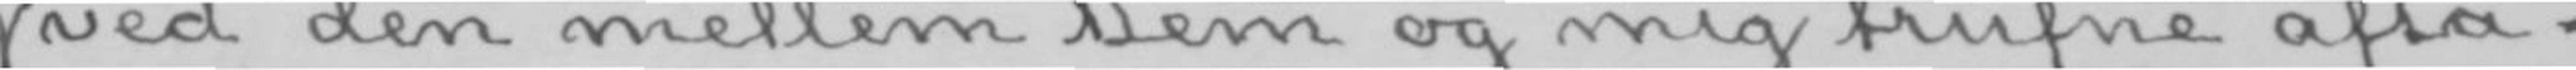ved den mellem Dem og mig trufne afta-
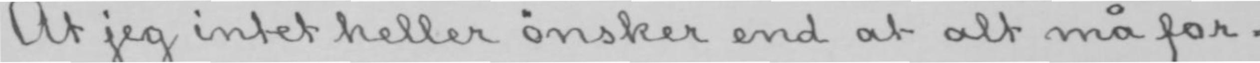At jeg intet heller ønsker end at alt må for-
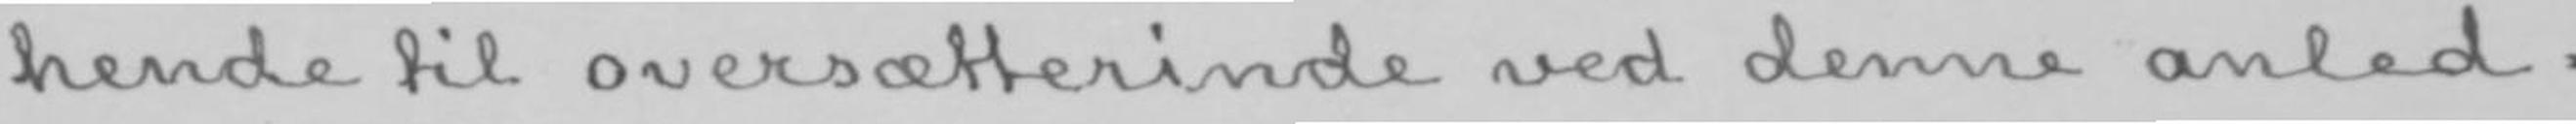hende til oversætterinde ved denne anled-
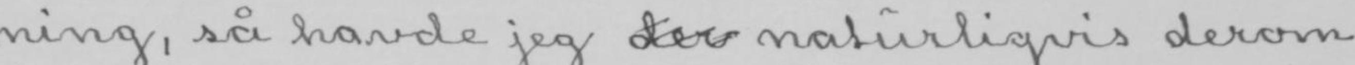ning, så havde jeg der naturligvis derom
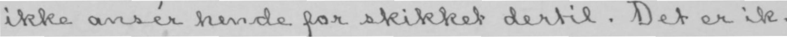ikke ansér hende for skikket dertil. Det er ik-
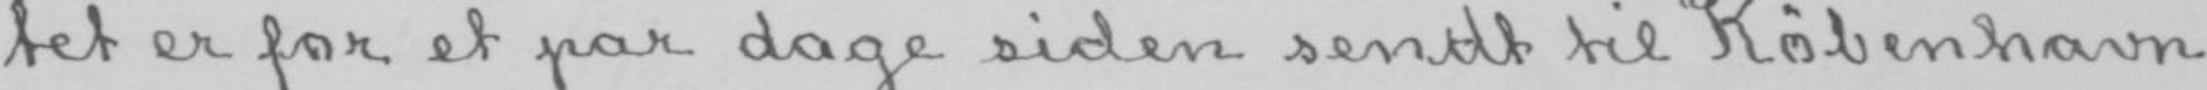tet er for et par dage siden sendt til København
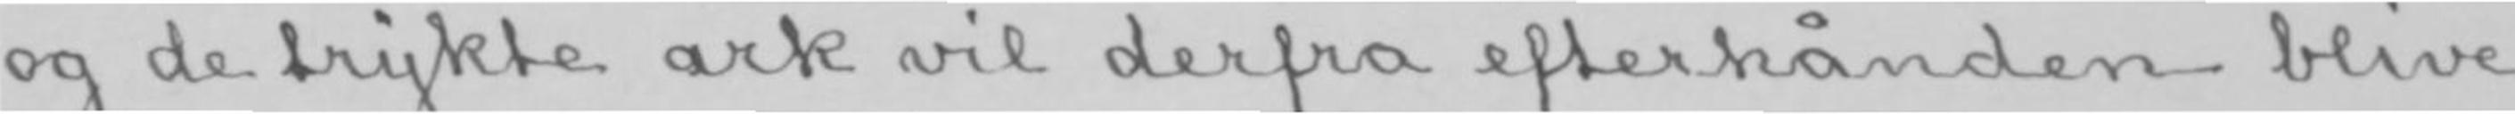og de trykte ark vil derfra efterhånden blive
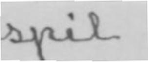spil.
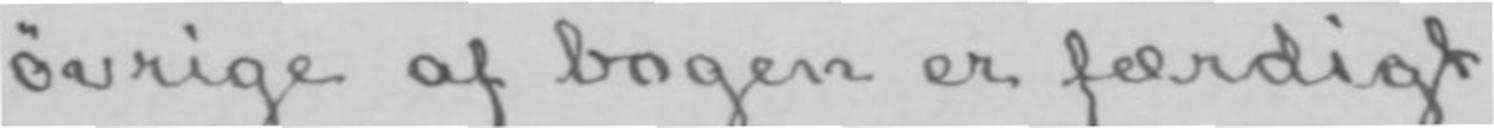øvrige af bogen er færdigt.
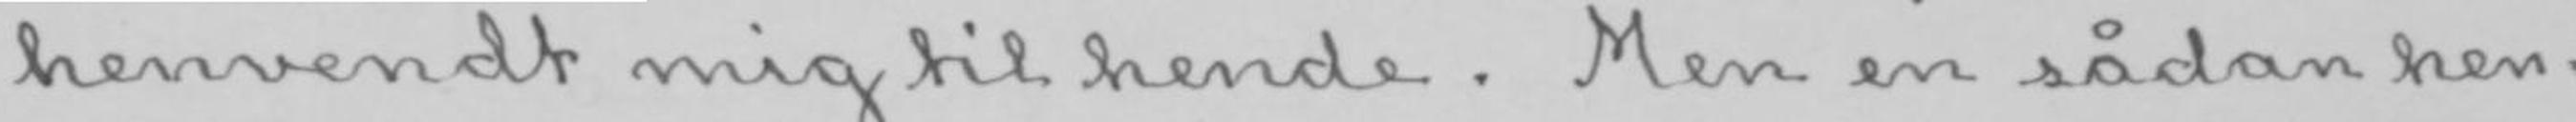henvendt mig til hende. Men en sådan hen-
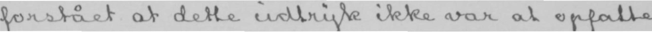forstået at dette udtryk ikke var at opfatte
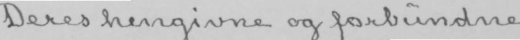Deres hengivne og forbundne
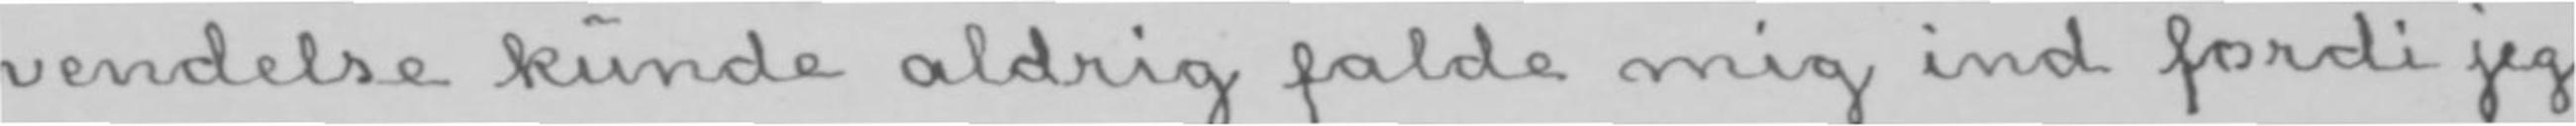vendelse kunde aldrig falde mig ind fordi jeg
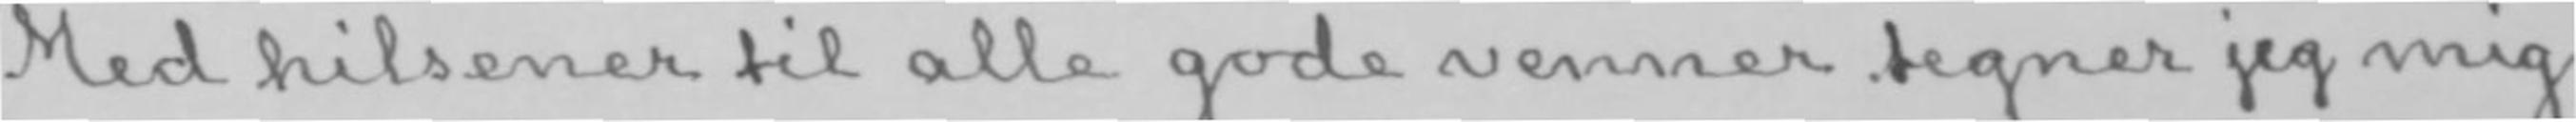Med hilsener til alle gode venner tegner jeg mig
